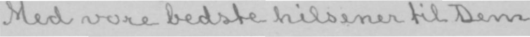Med vore bedste hilsener til Dem
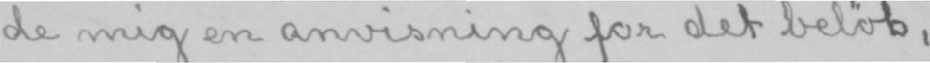de mig en anvisning for det beløb,
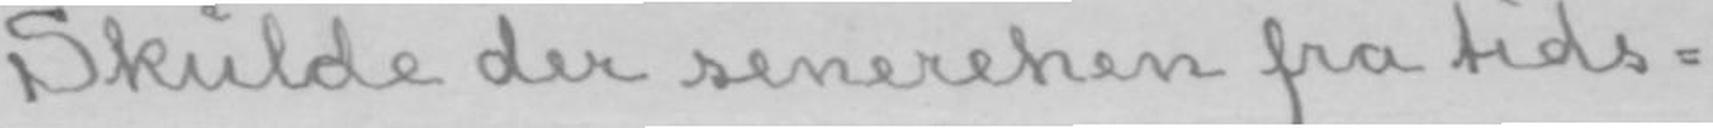Skulde der senerehen fra tids-
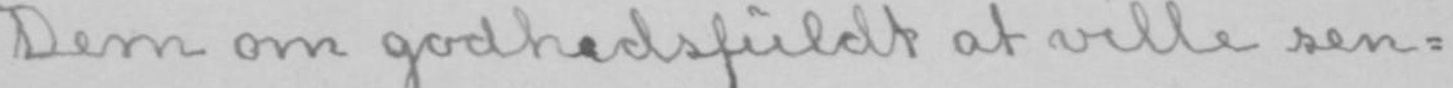Dem om godhedsfuldt at ville sen-
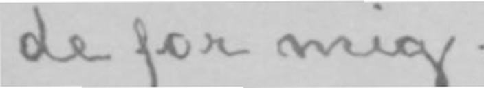de for mig.
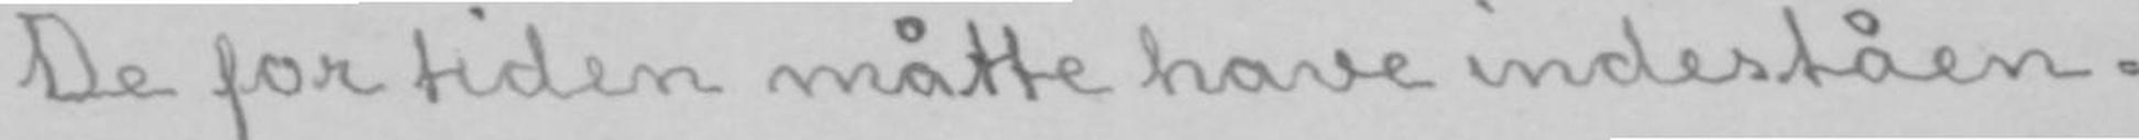De for tiden måtte have indeståen-
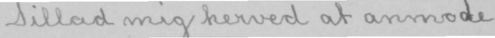Tillad mig herved at anmode
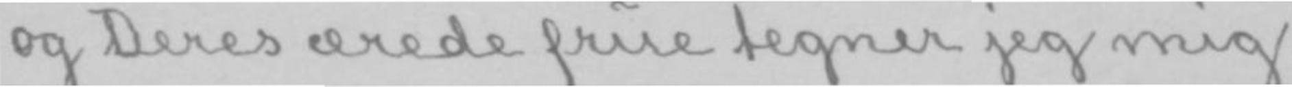og Deres ærede frue tegner jeg mig
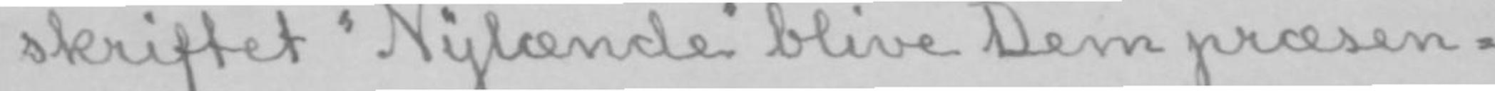skriftet «Nylænde» blive Dem præsen-
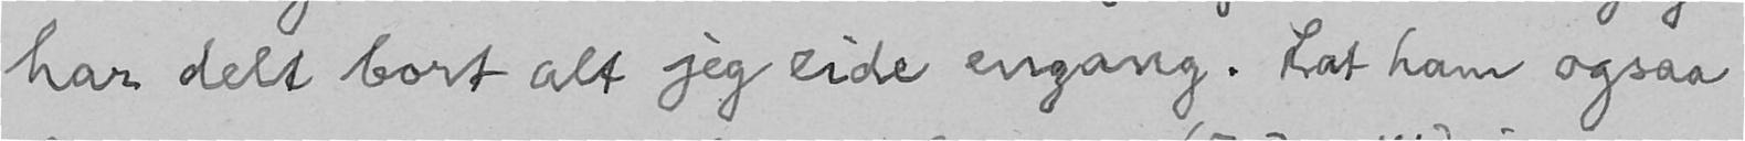har delt bort alt jeg eide engang. Lat ham ogsaa
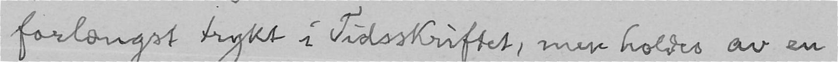forlængst trykt i Tidsskriftet, men holdes av en
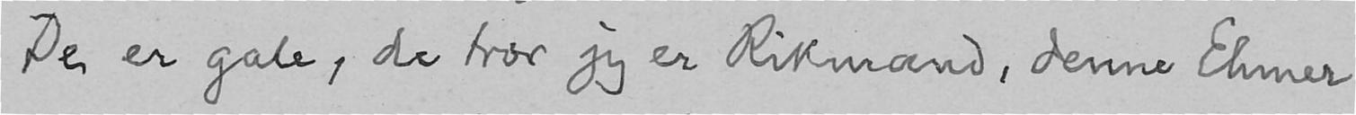De er gale, de tror jeg er Rikmand, denne Ehmer
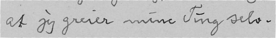at jeg greier mine Ting selv.
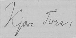Kjære Tore,
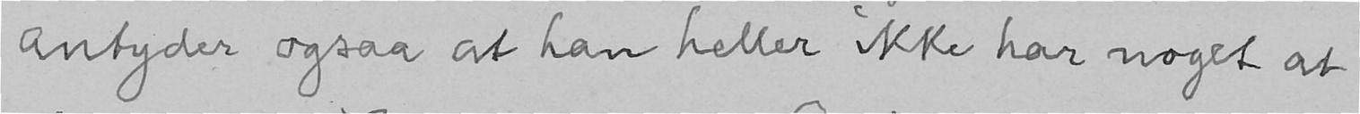antyder ogsaa at han heller ikke har noget at
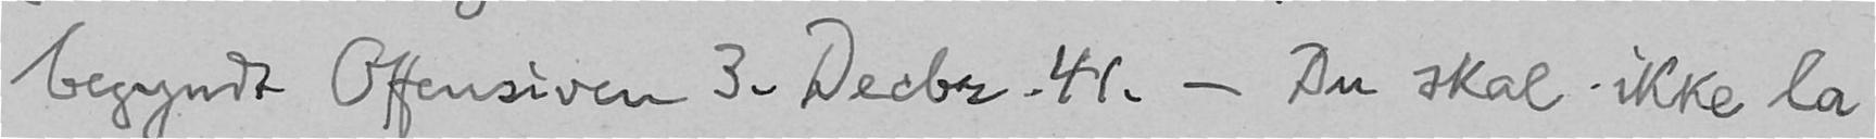begyndt Offensiven 3. Decbr. 41. - Du skal ikke la
